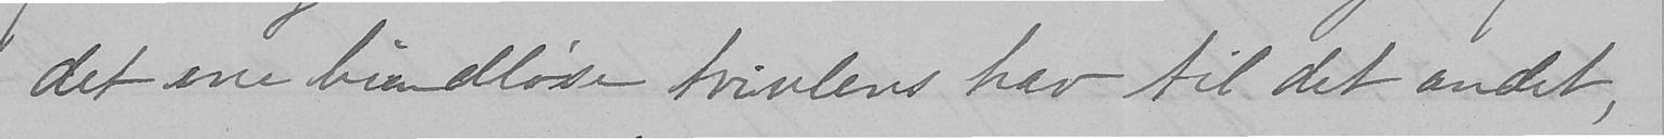det ene bundløse tvivlens hav til det andet,
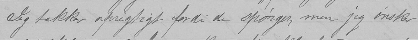Jeg takker oprigtigt fordi de spørger, men jeg ønsker
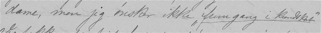dame, men jeg ønsker ikke "fremgang i kundskab."
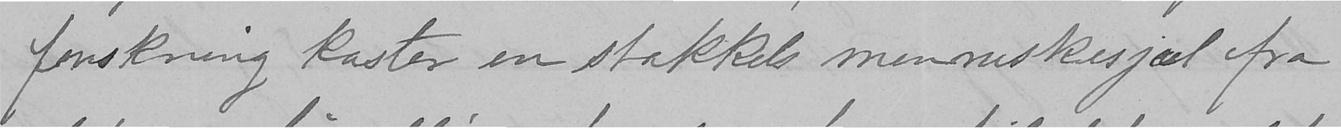forskning kaster en stakkels menneskesjæl ifra
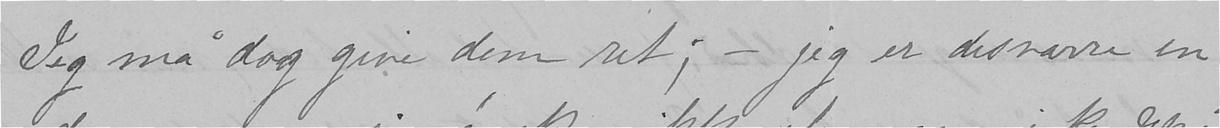Jeg må dog give dem ret; - jeg er desværre en
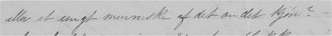eller et ungt menneske af det andet kjøn? –
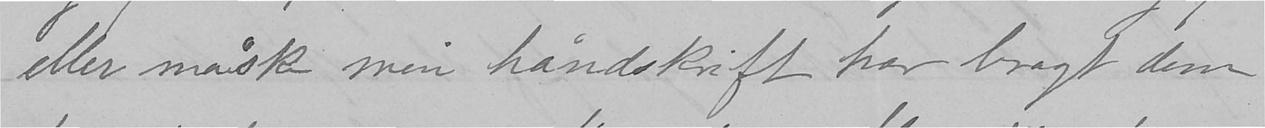eller måske min håndskrift har bragt dem
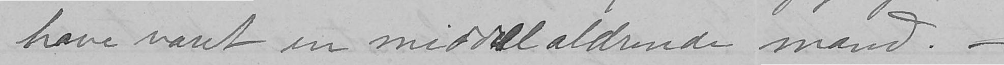have været en middalaldrende mand. -
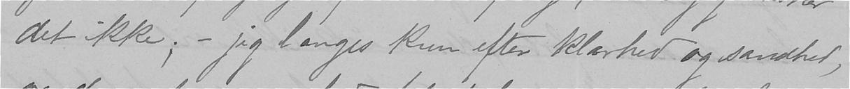det ikke; - jeg længes kun efter klarhed og sandhed,
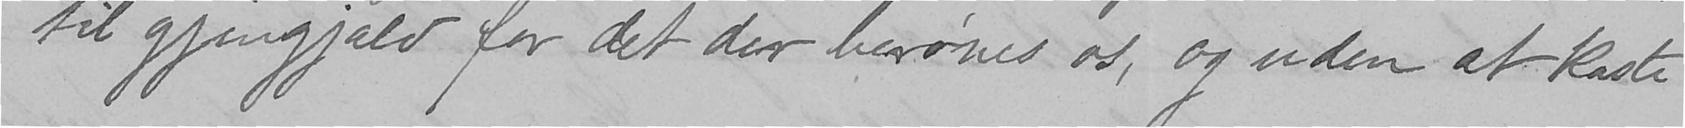til gjengjæld for det der berøves os, og uden at kaste
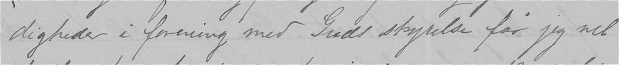digheder i forening med Guds skyrelse får jeg vel
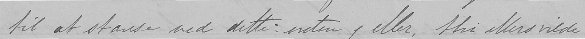til at stanse ved dette: enten/eller, thi ellers vilde
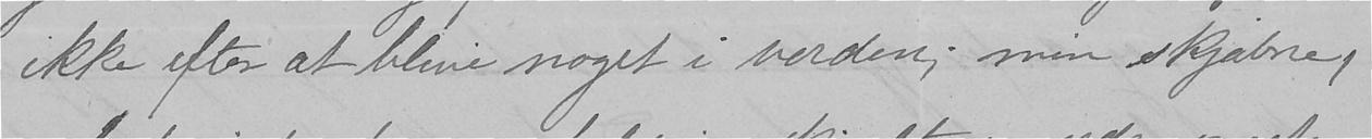ikke efter at blive noget i verden; min skjæbne,
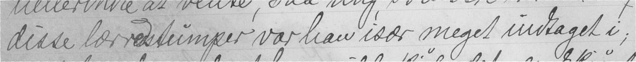disse lærredstumper var han især meget indtaget i;
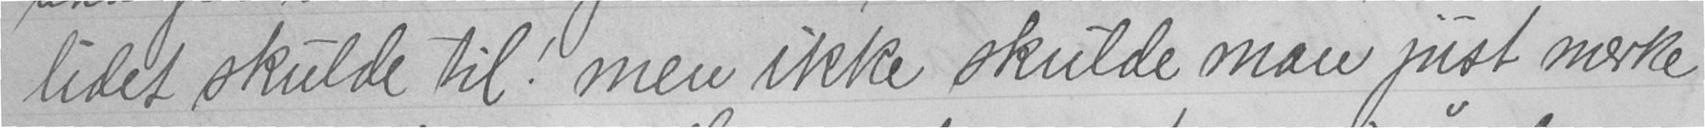lidet skulde til! men ikke skulde man just merke
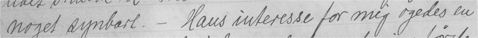noget synbart. - Hans interesse for mig øgedes en
